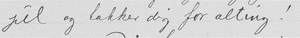jul og takker dig for alting!
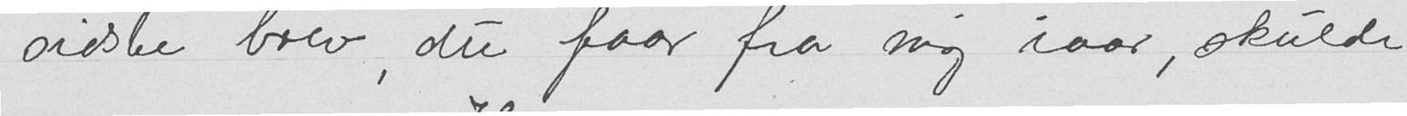sidste brev, du faar fra mig iaar, skulde
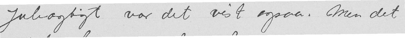Juleagtigt var det vist ogsaa. Men det
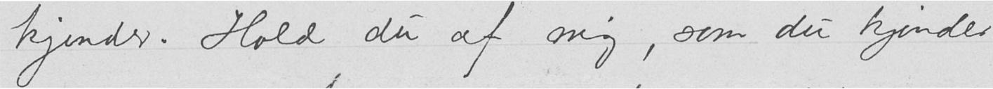kjender. Hold du af mig, som du kjender
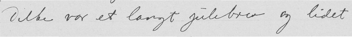Dette var et langt julebrev og lidet
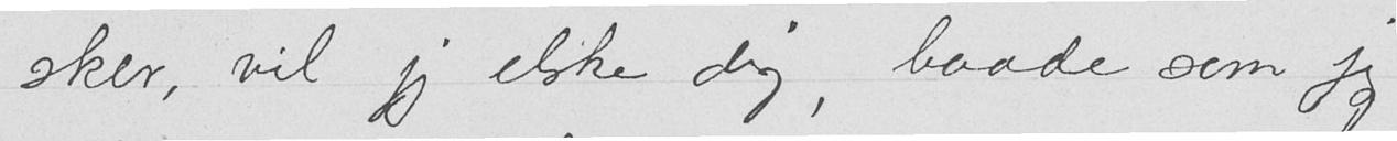sker, vil jeg elske dig, baade som jeg
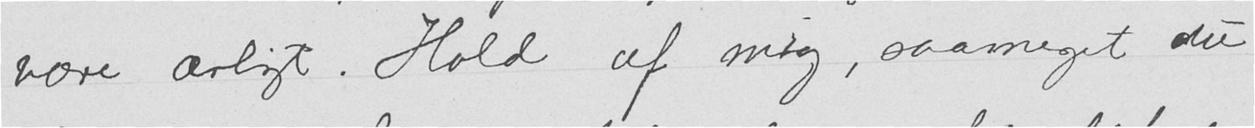være ærligt. Hold af mig, saameget du
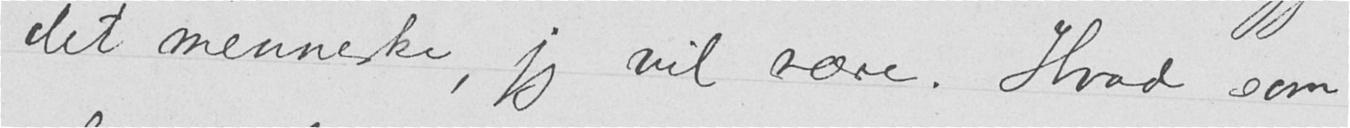det menneske, jeg vil være. Hvad som
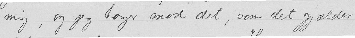mig, og jeg tager mod det, som det gjælder
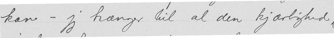kan - jeg hænger til al den kjærlighed,
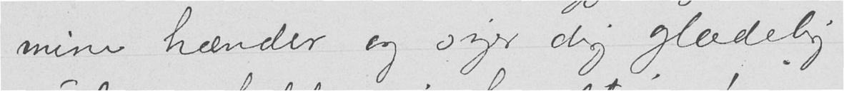mine hænder og siger dig glædelig
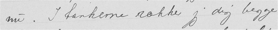nu. I tankerne rækker jeg dig begge
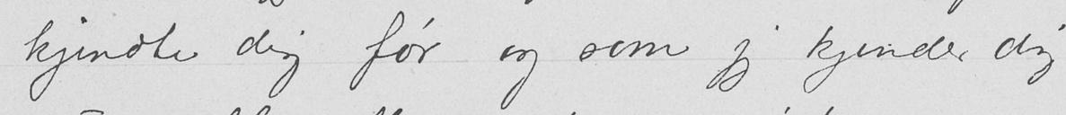kjendte dig før og som jeg kjender dig
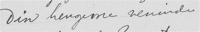Din hengivne veninde
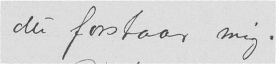du forstaar mig.
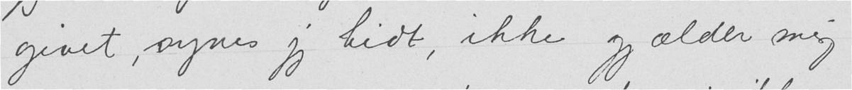givet, synes jeg tidt, ikke gjælder mig
